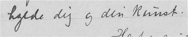hylde dig og din kunst.
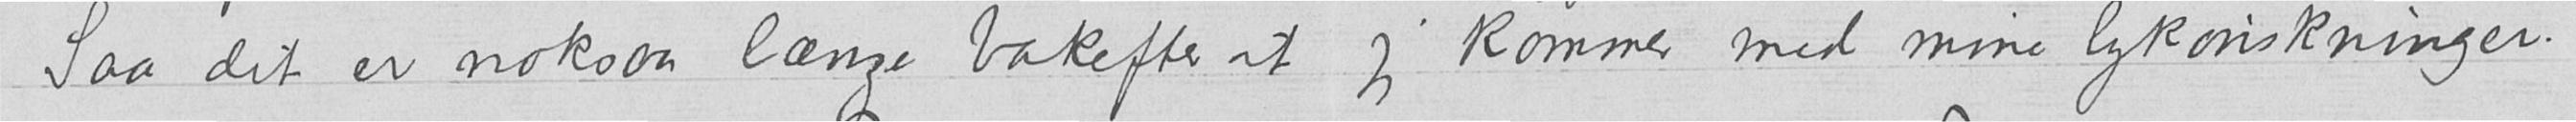Saa det er noksaa længe bakefter at jeg kommer med mine lykønskninger.
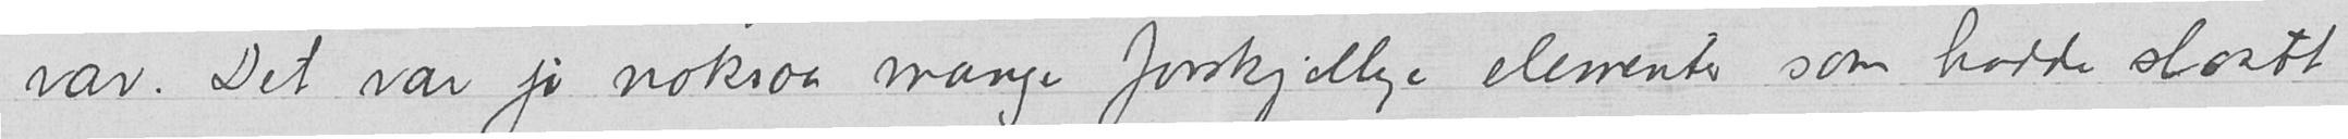var. Det var jo noksaa mange forskjellige elementer som hadde slaatt
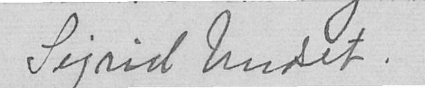Sigrid Undset.
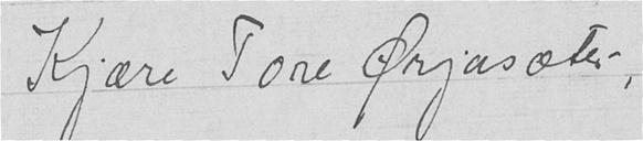Kjære Tore Ørjasæter,
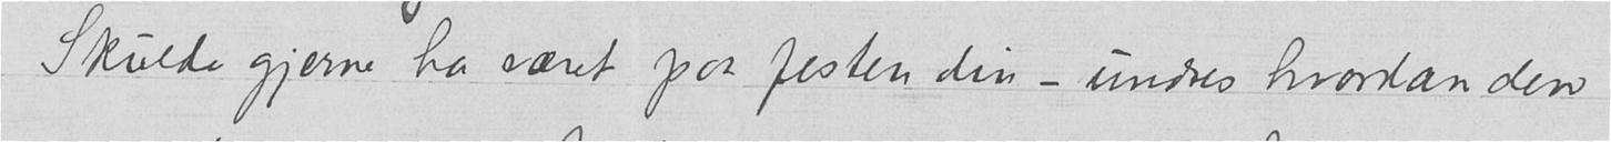Skulde gjerne ha været paa festen din - undres hvordan den
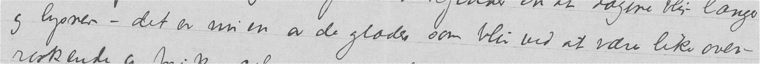og lysner – det er nu en av de glæder som blir ved at være like over-
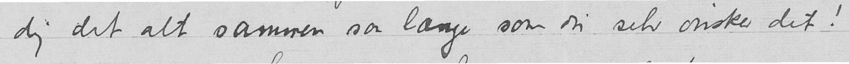dig det alt sammen saa længe som du selv ønsker det!
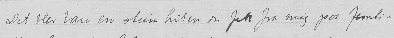Det blev bare en stum hilsen du fik fra mig paa femti-
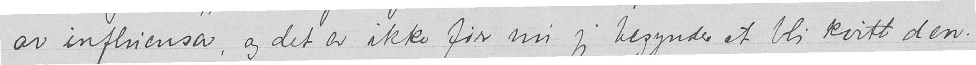av influensa, og det er ikke før nu jeg begynder at bli kvitt den.
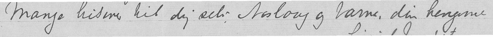Mange hilsener til dig selv, Aaslaug og barna, din hengivne
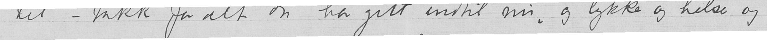til" – takk for alt du har gitt indtil nu, og lykke og helse og
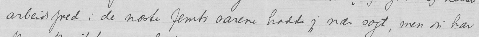arbeidsfred i de næste femti aarene hadde jeg nær sagt, men du har
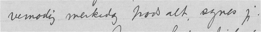vemodig merkedag trods alt, synes jeg.
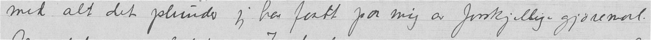med alt det plunder jeg har faatt paa mig av forskjellige gjøremaal.
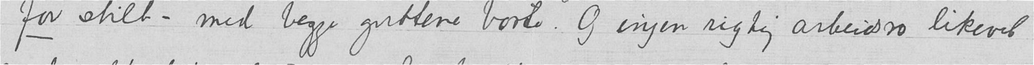- for stilt – med begge guttene borte. Og ingen rigtig arbeidsro likevel
